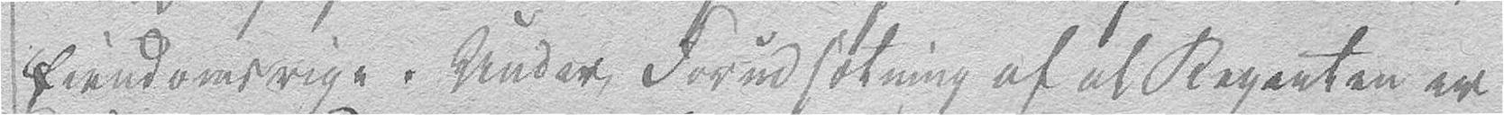Eiendomsrige. Under Forudsætning af at Regenten er
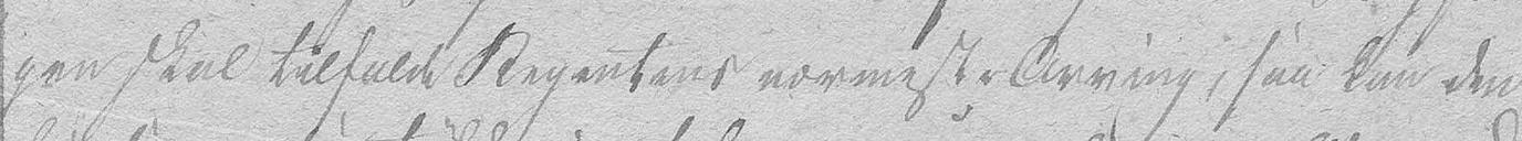gen skal tilfalde Regentens nærmeste Arving, saa kan den
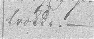trække.–
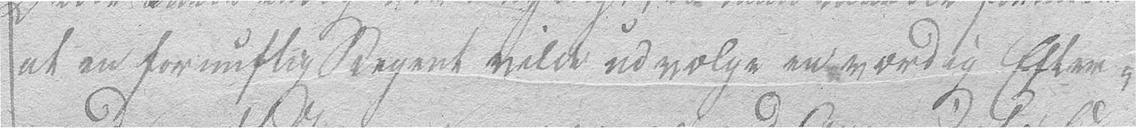at en fornuftig Regent vilde udvælge en værdig Efter-
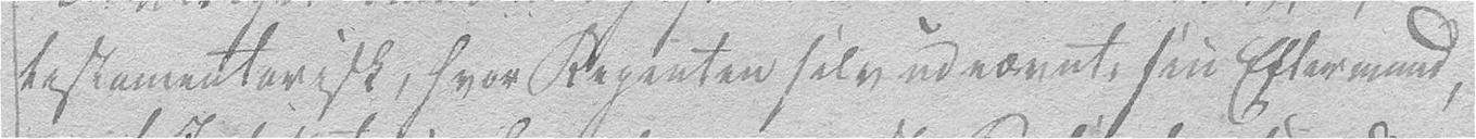testamentarisk, hvor Regenten selv udnævnte sin Eftermand,
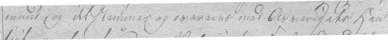mand og det stemmer og overeens med Arverigets Hen-
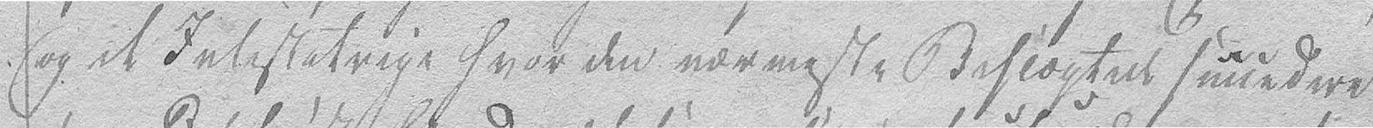og et Intestatrige hvor den nærmeste Beslægtede succedere-
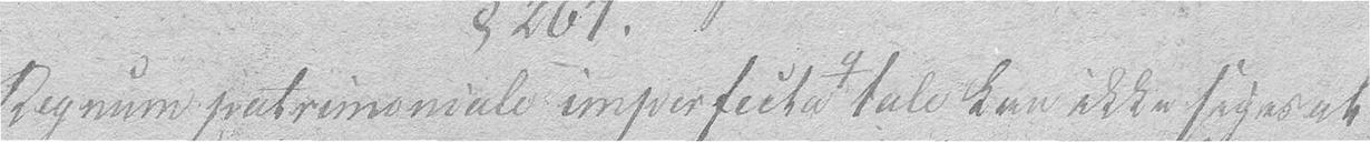Regnum patrimoniale imperfecta tale kan ikke siges at
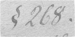§ 268.
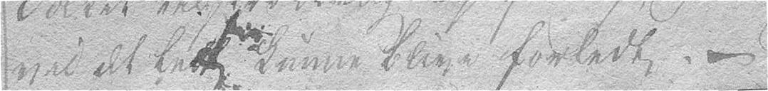vil det let kunne blive forledt.–
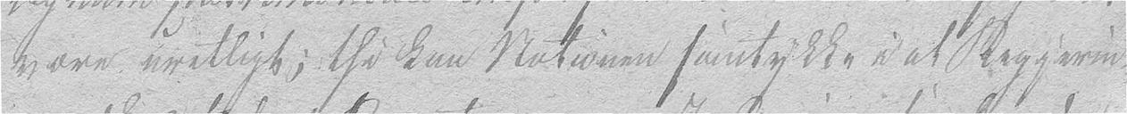være uretligt; thi kan Nationen samtykke i at Regjerin-
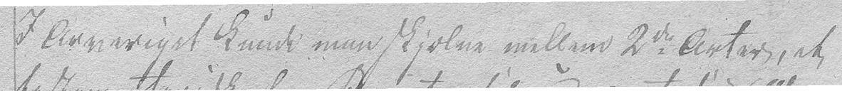I Arveriget kunde man skjælne mellem 2de Arter, et
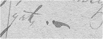get.–
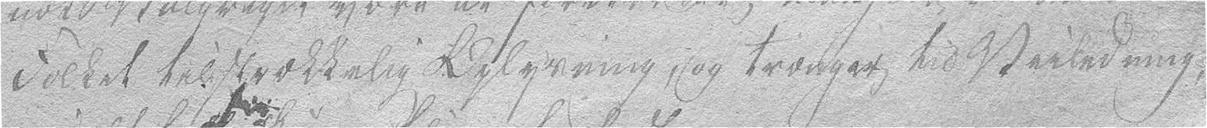Folket tilstrækkelig Oplysning, og trænger til Veiledning,
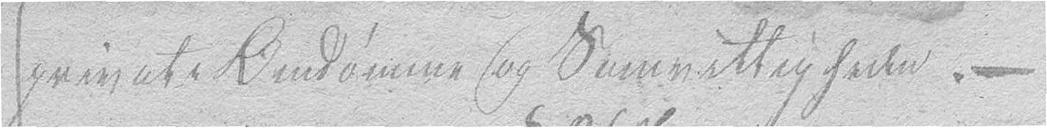private Omdømme og Samvittigheden.–
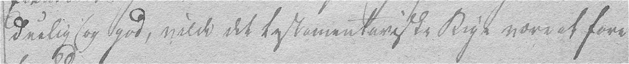duelig og god, vilde det testamentariske Rige være at fore-
